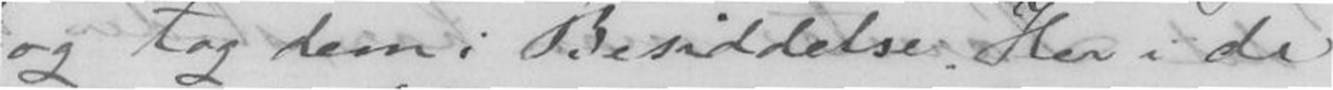og tog dem i Besiddelse. Her i de
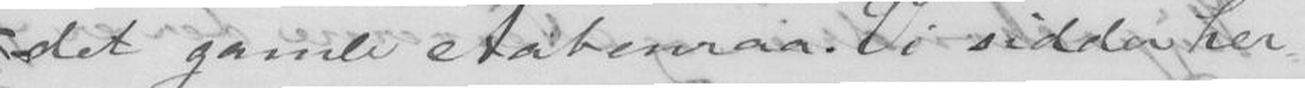det gamle Aabenraa. Vi sidder her
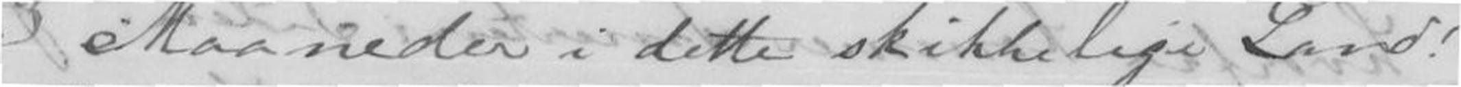3 Maaneder i dette skikkelige Land!
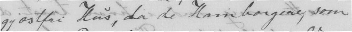gjæstfri Hus, da de Hamborgere, som
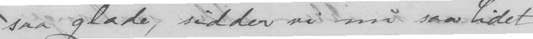saa glade, sidder vi nu saa Lidet
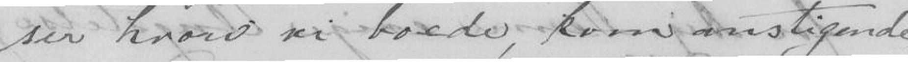ser hvori vi boede, kom anstigende
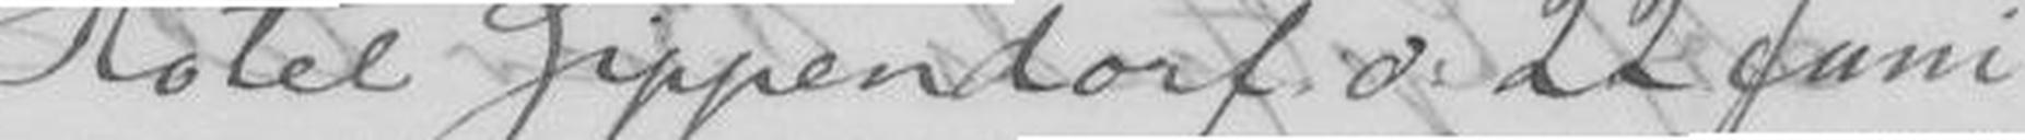Hotel Zippendorf d. 22 Juni
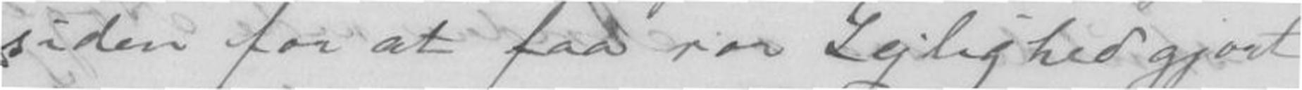siden for at faa vor Leilighed gjort
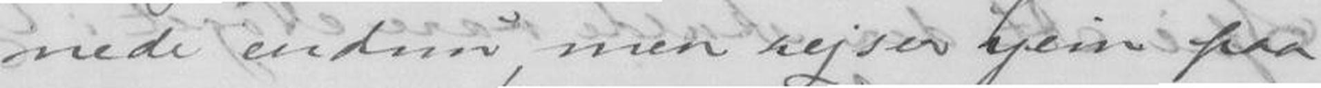nede endnu, men rejser hjem paa
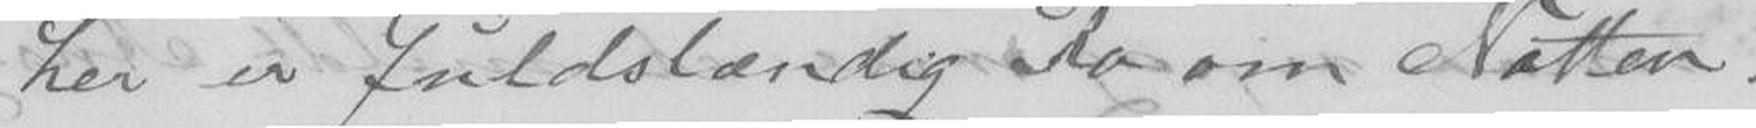her er fuldstændig Ro om Natten.
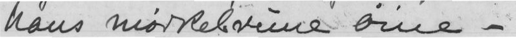hans mørkebrune øine -
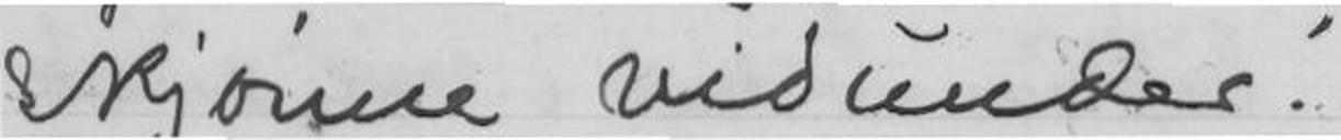skjønne vidunder!
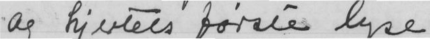og hjertets første lyse
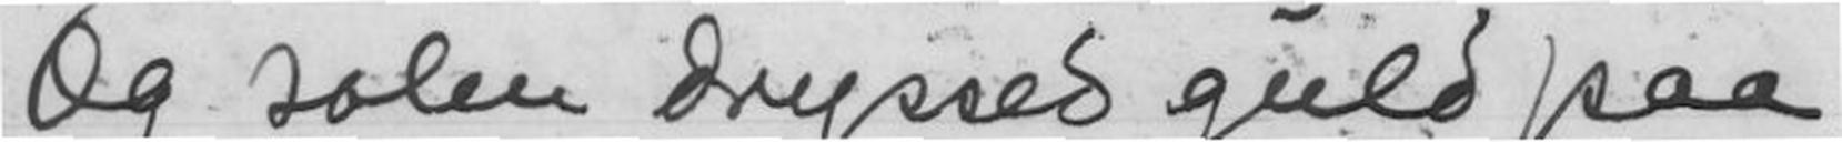Og solen drysses guld paa
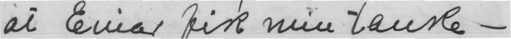at Einar fik min tanke -
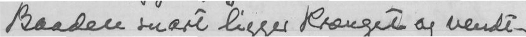Baaden snart ligger krænget og vendt -
